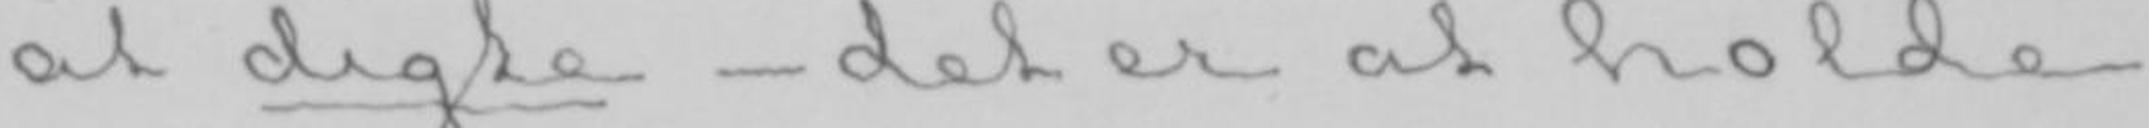at digte - det er at holde
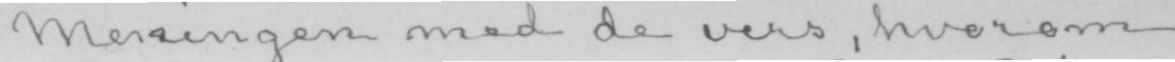Meningen med de vers, hvorom
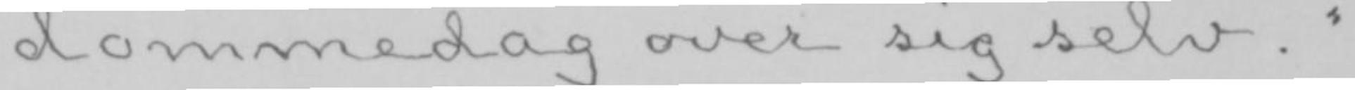dommedag over sig selv.»
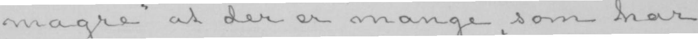magre» at der er mange, som har
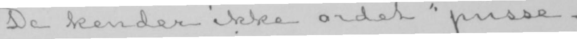De kender ikke ordet «pusse-
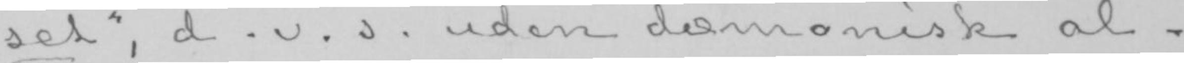set», d. v. s. uden dæmonisk al-
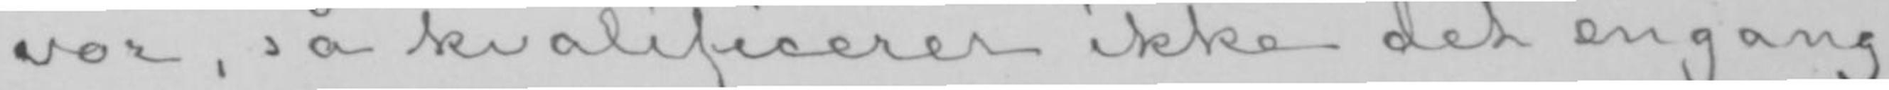vor, så kvalificerer ikke det engang
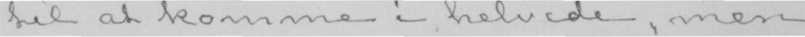til at komme i helvede, men
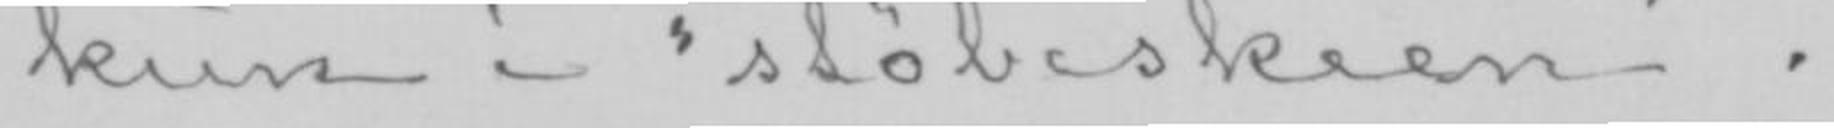kun i «støbeskeen».
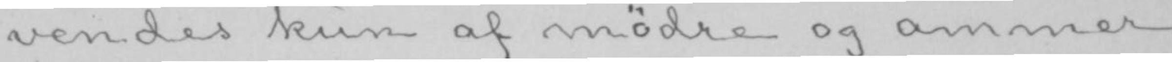vendes kun af mødre og ammer
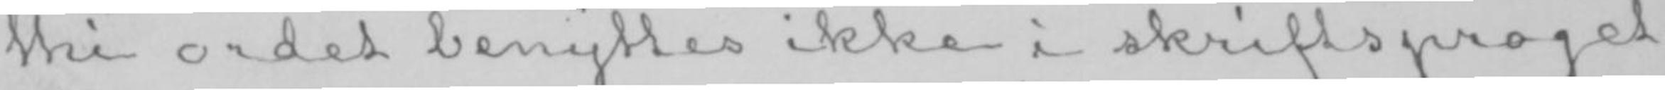thi ordet benyttes ikke i skriftsproget,
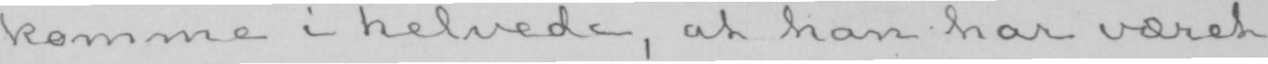komme i helvede, at han har været
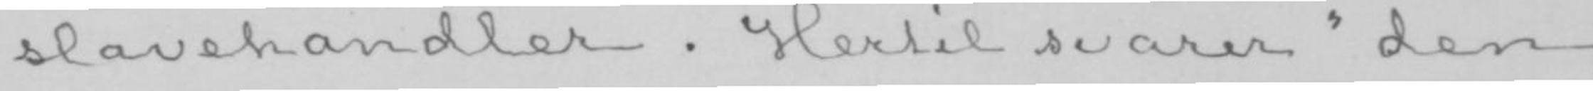slavehandler. Hertil svarer «den
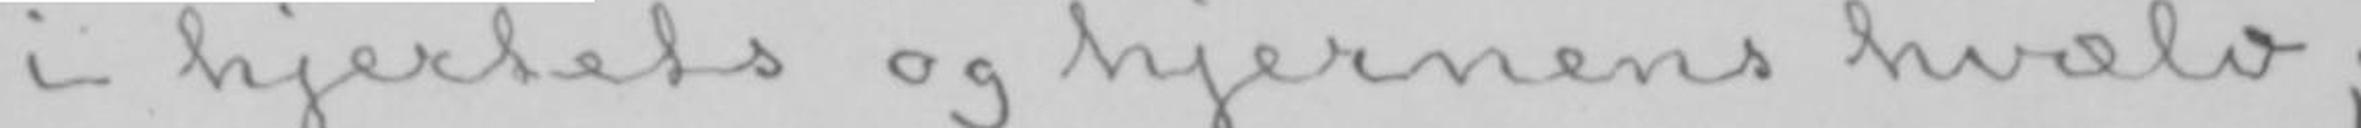i hjertets og hjernens hvælv;
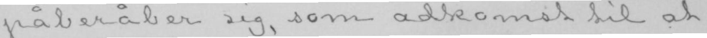påberåber sig, som adkomst til at
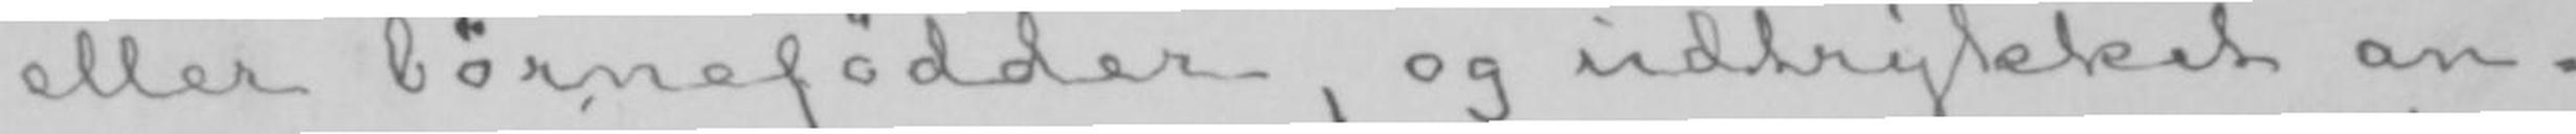eller børnefødder, og udtrykket an-
